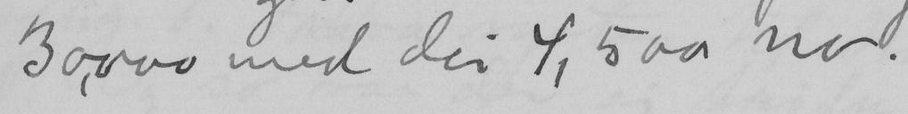30,000 med dei 4,500 no.
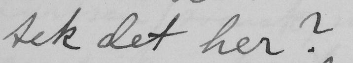tek det her?
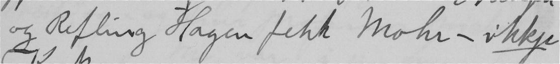og Refling Hagen fekk Mohr – ikkje
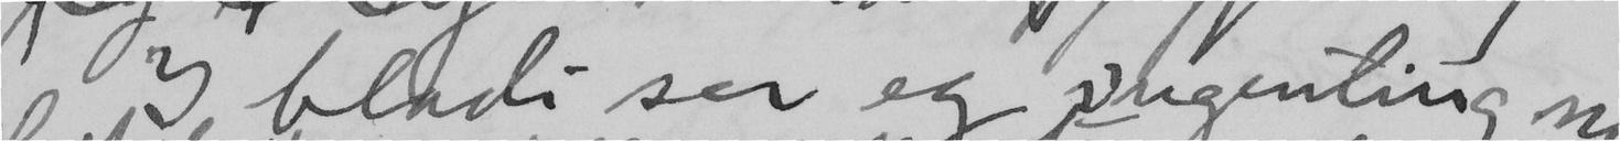Og bladi ser eg ingenting no.
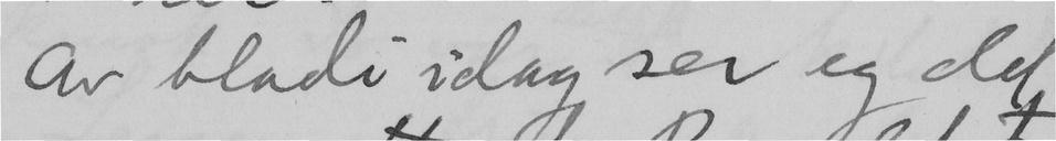Av bladi idag ser og det
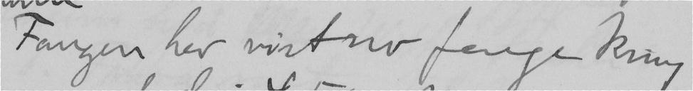Fangen hev vistno fenge kring
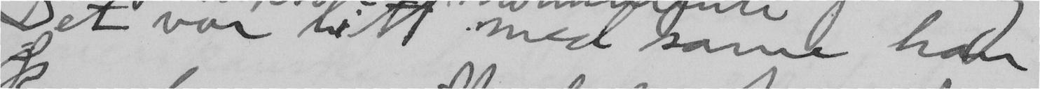Det var litt med same han
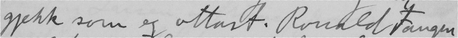gjekk som eg ottast. Ronald Fangen
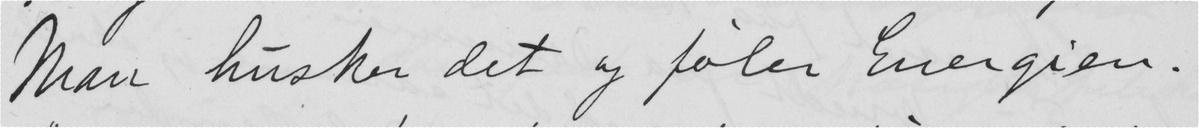Man husker det og føler Energien.
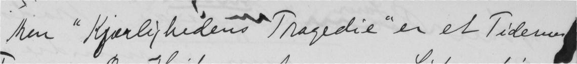Men "Kjærlighedens Tragedie" er et Tidernes
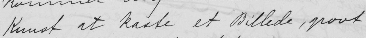Kunst at kaste et Billede, grovt
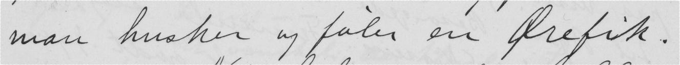man husker og føler en Ørefik.
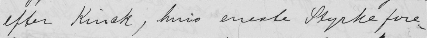efter Kinck, hvis eneste Styrke fore-
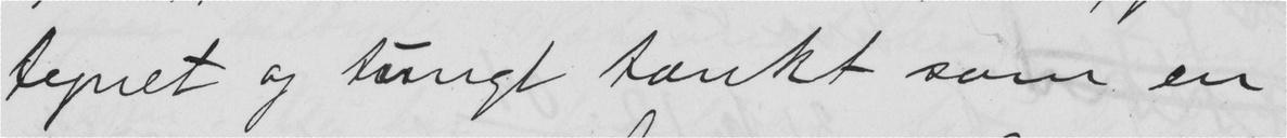tegnet og tungt tænkt som en
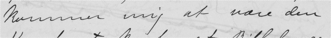kommer mig at være den han
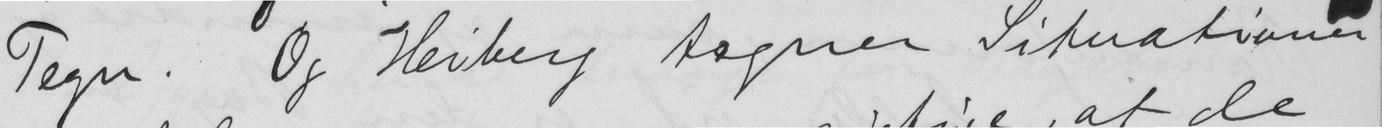Tegn. Og Heiberg tegner Situationer
